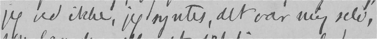jeg ved ikke, jeg syntes, det var mig selv,
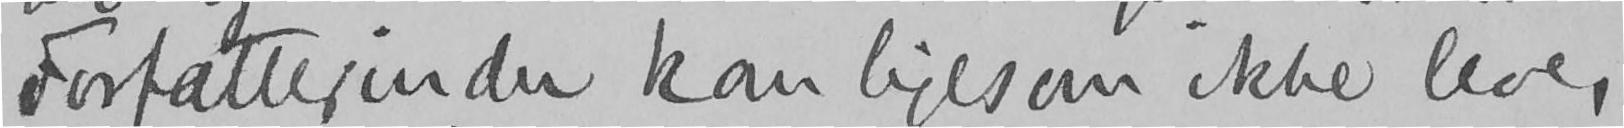Forfatterinder kan ligesom ikke leve,
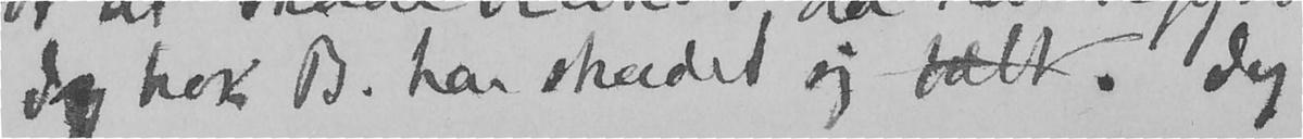Jeg tror B. har skadet sig fælt. Jeg
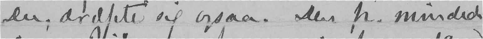Du, dræpete sig ogsaa. Den fru mindede
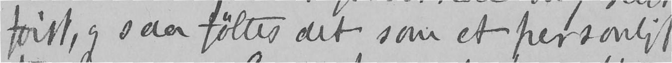først, og saa føltes det som et personligt
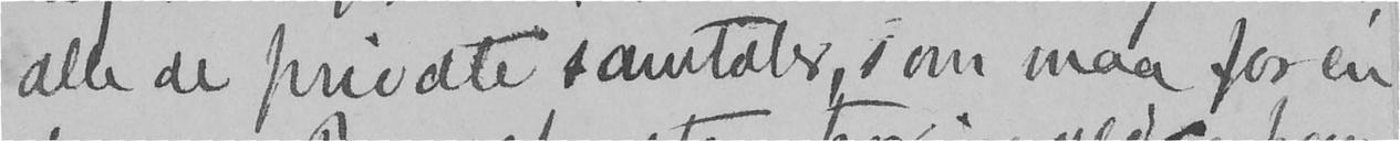alle de private samtaler, som maa for en
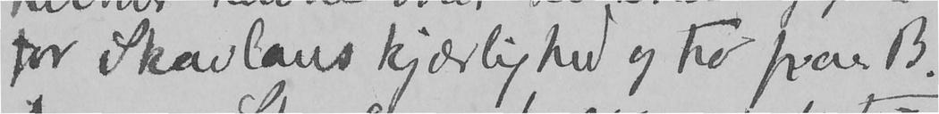for Skavlans kjærlighed og tro paa B.
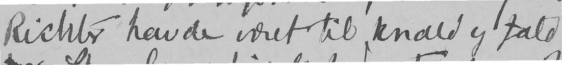Richter havde været til knald og fald
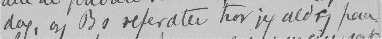dag, og Bs referater tror jeg aldrig paa.
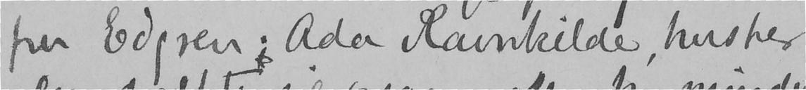fru Edgren. Ada Ravnkilde, husker
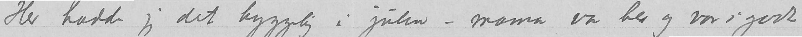Her hadde jeg det hyggelig i julen – mama var her og var i godt
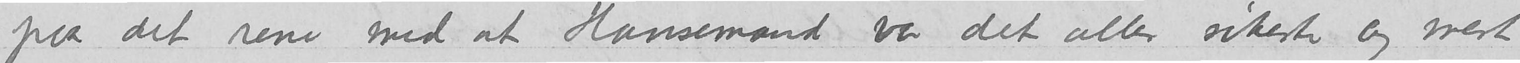paa det rene med at Hansemand var det aller søteste og mest
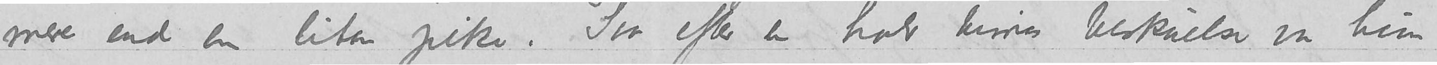litt mere end en liten pike.  Saa efter en halv times beskuelse var hun
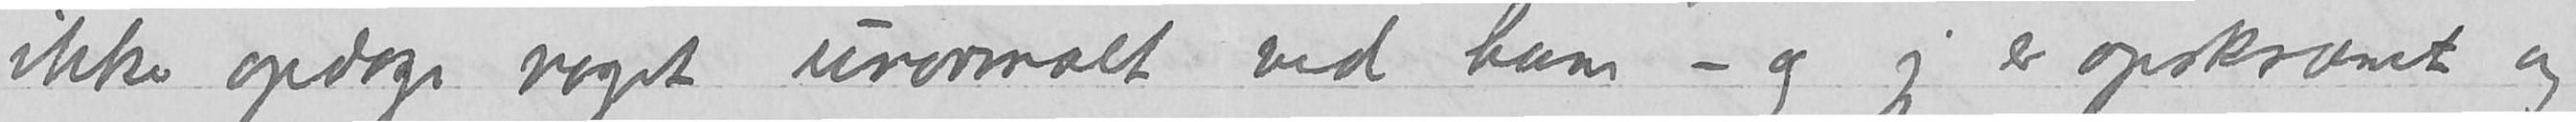ikke opdage noget unormalt ved ham – og jeg er opskræmt og
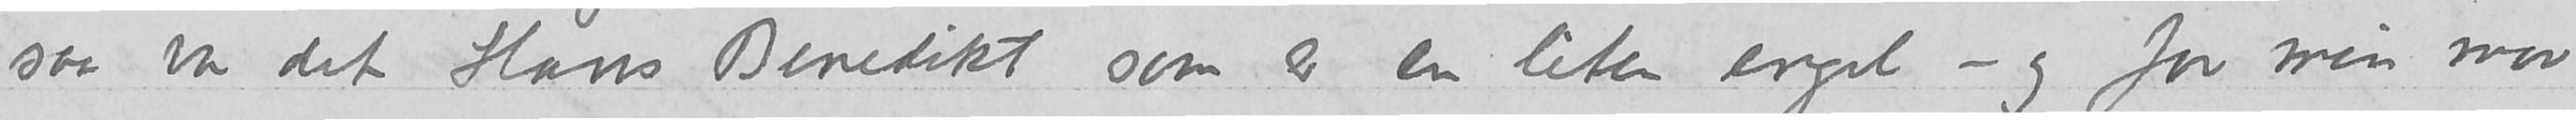var det Hans Benedikt som er en liten engel – og for min mor
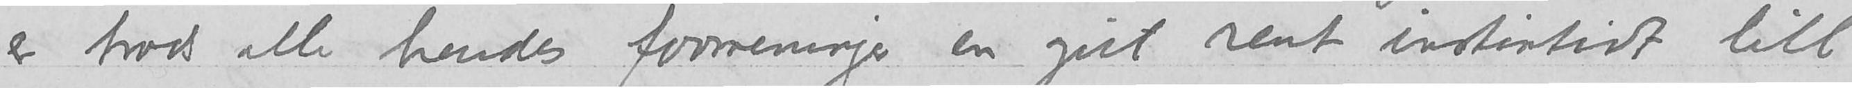er trods alle hendes formeninger en gut rent instinktivt litt
